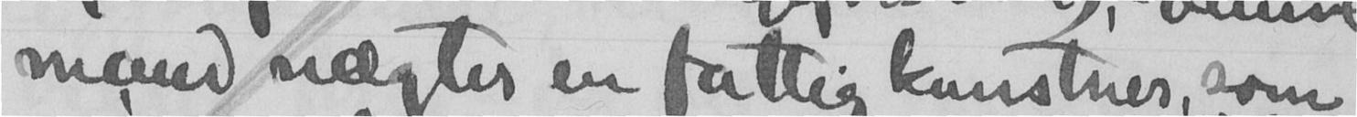mand nægter en fattig kunstner, som
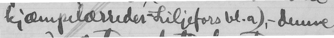kjæmpelærreder-Liljefors bl.a), - denne
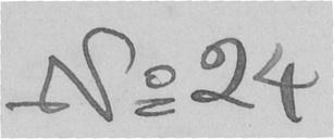No 24
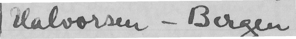Halvorsen - Bergen
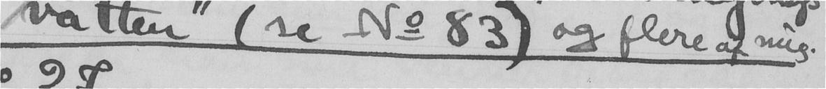vatten" (se No 83) og flere af mig.
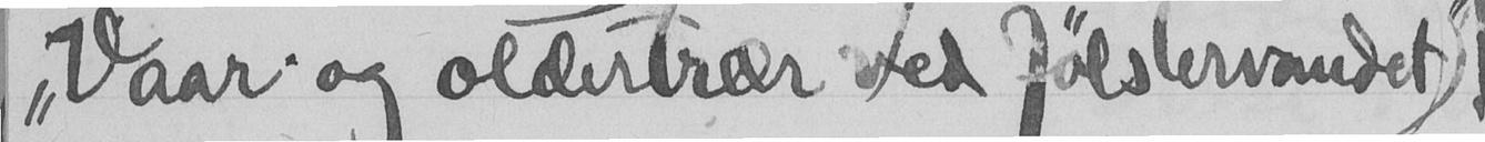"Vaar og oldertrær ved Jølstervandet")
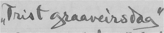"Trist graaveirsdag"
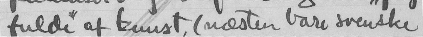fulde af kunst, (næsten bare svenske
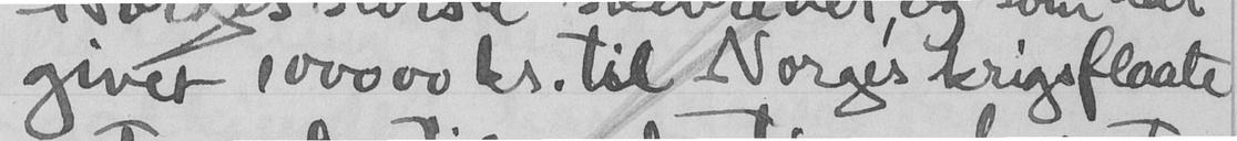givet 100 000 kr. til Norges krigsflaate
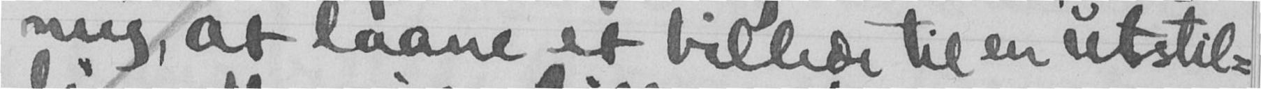mig, at laane et billede til en utstil-
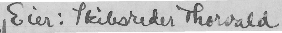Eier: Skibsreder Thorvald
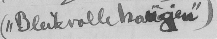("Bleikvollehaugen")
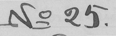No 25.
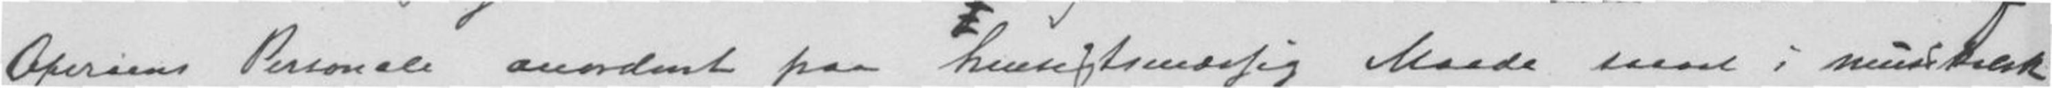Operaens Personale anordent paa baade mod i
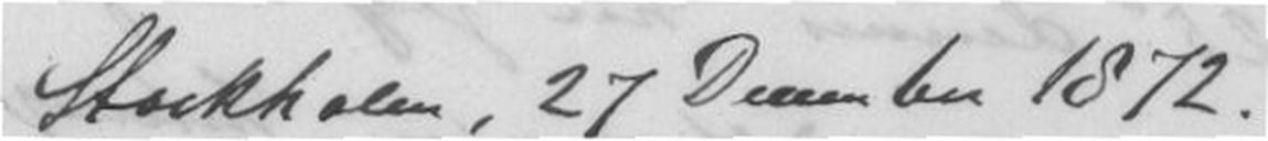Stockholm, 27 December 1872
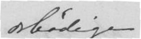ærbødige
